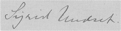Sigrid Undset.
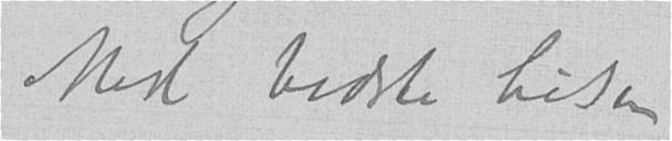Med bedste hilsen
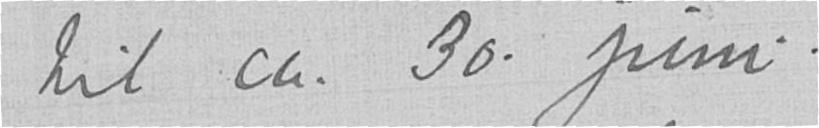til ca. 30. juni.
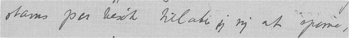stams paa besøk tillater jeg mig at spørre,
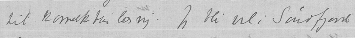til korrektur hos mig. Jeg blir vel i Sundfjord
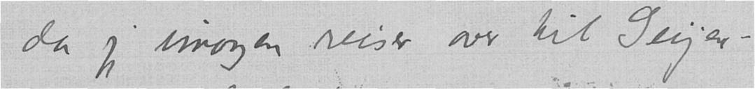da jeg imorgen reiser over til Geijer-
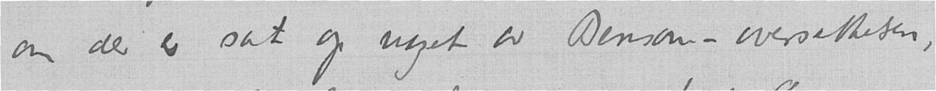om der er sat op noget av Benson-oversettelsen,
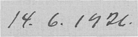14.6.1926.
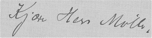Kjære Herr Møller,
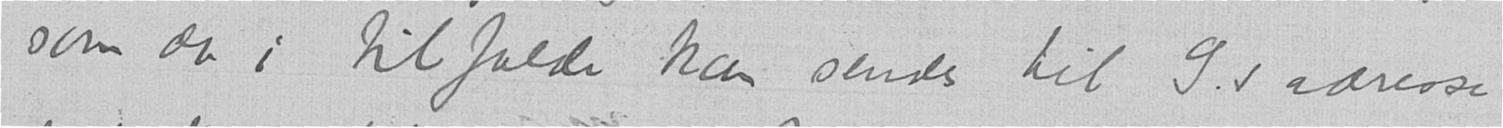som der i tilfælde kan sendes til G.s adresse
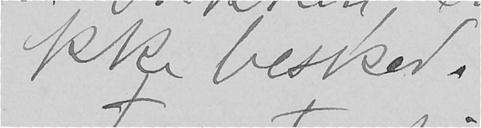ikke besked.
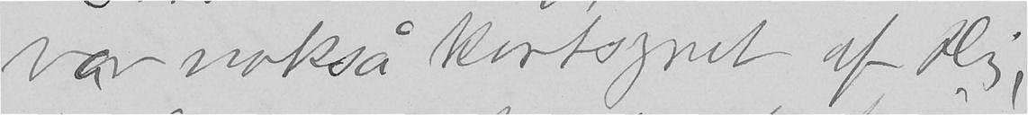var nokså kortsynet af Dig,
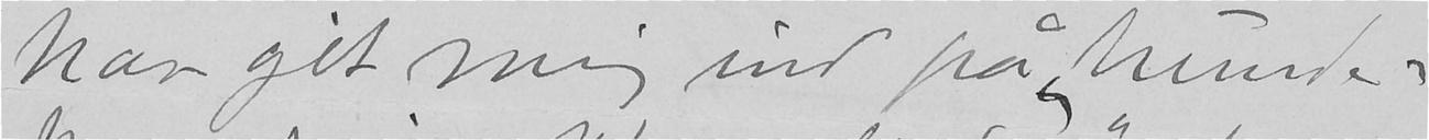har git mig ind på "hunde-
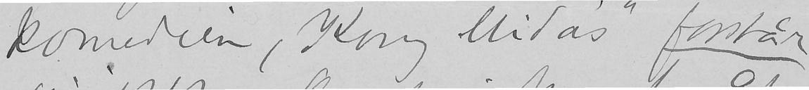komedien, Kong Midas" forstår
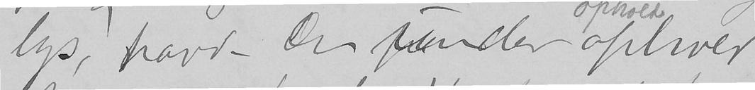lys, havde Du under ophold
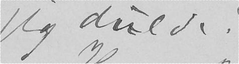jeg duede.
